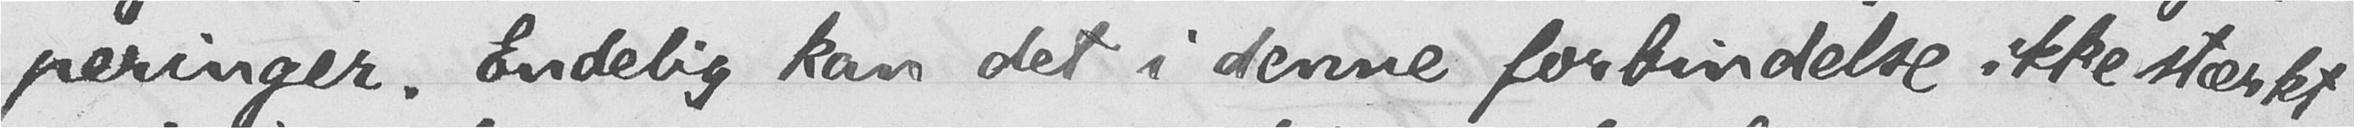peringer. Endelig kan det i denne forbindelse ikke stærkt
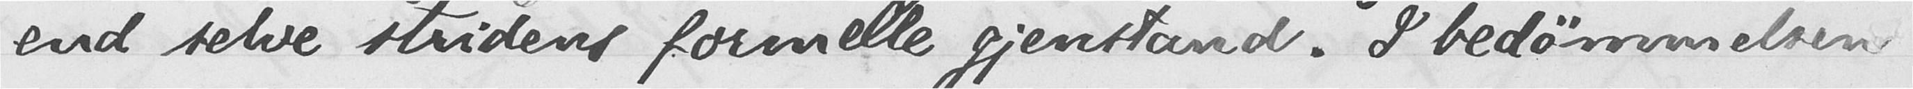end selve stridens formelle gjenstand. I bedømmelsen
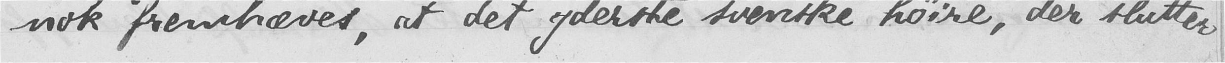nok fremhæves, at det yderste svenske høire, der slutter
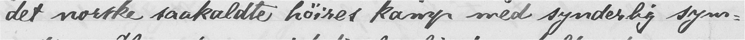det norske saakaldte høires kamp med synderlig sym-
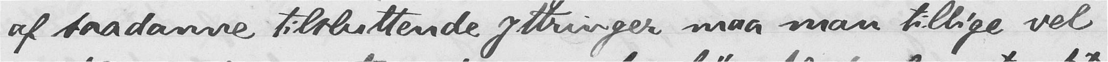af saadanne tilsluttende yttringer maa man tillige vel
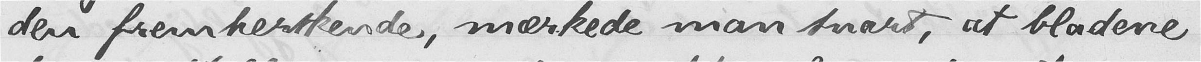den fremherskende, mærkede man snart, at bladene
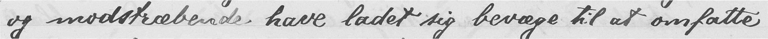og modstræbende have ladet sig bevæge til at omfatte
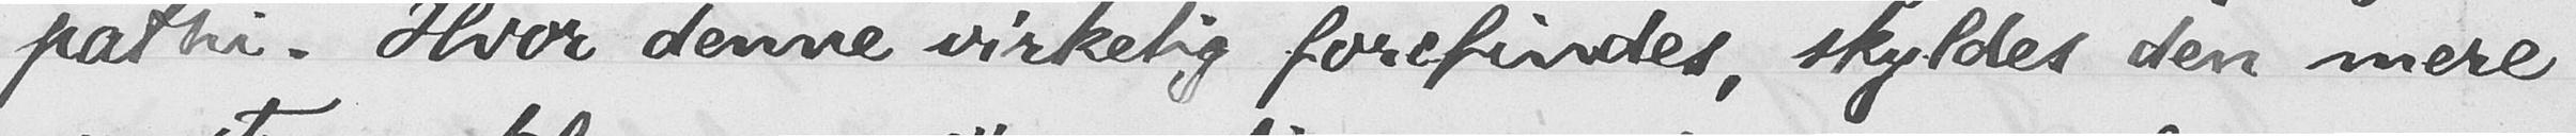pathi. Hvor denne virkelig forefindes, skyldes den mere
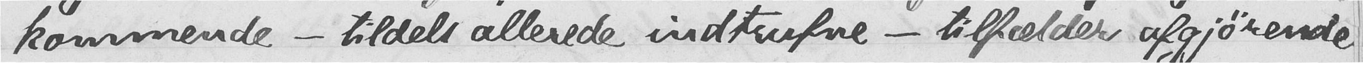kommende - tildels allerede indtrufne - tilfælder afgjørende
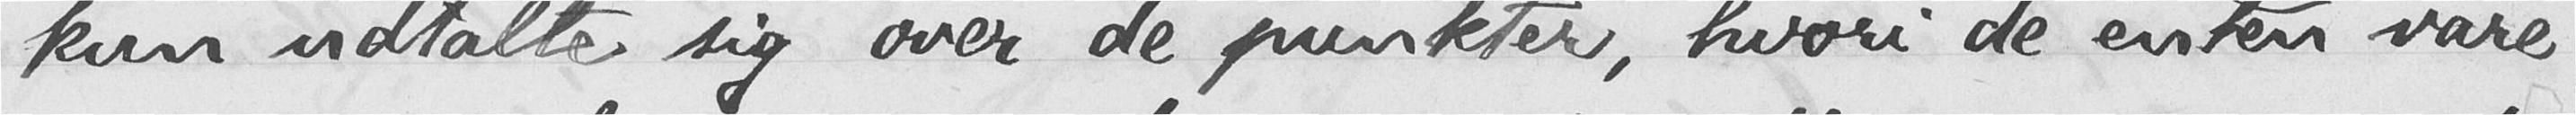kun udtalte sig over de punkter, hvori de enten vare
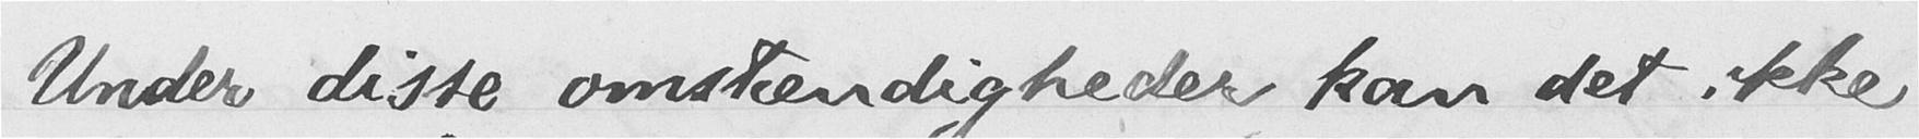Under disse omstændigheder kan det ikke
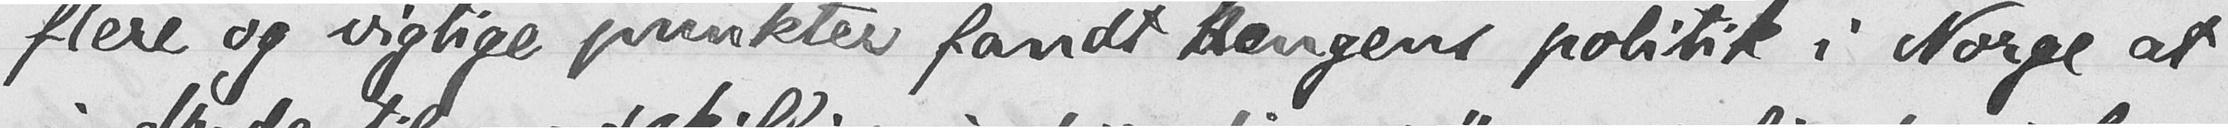flere og vigtige punkter fandt kongens politik i Norge at
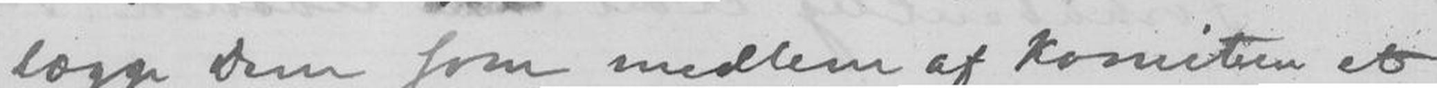lægge dem som medlem af komiteen et
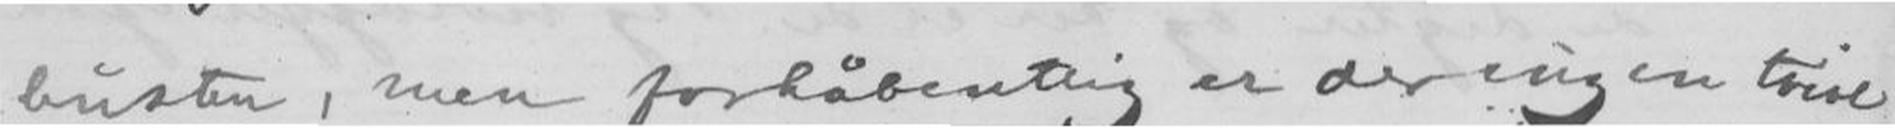busten, men forhåbentlig er der ingen krise
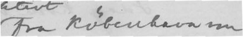fra København om
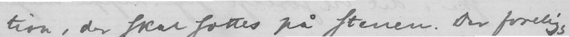tion, der skal fattes på stenen. Der forelig-
